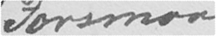Forsmaa
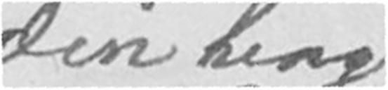din heng
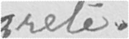grete.
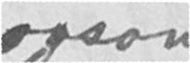ogsaa
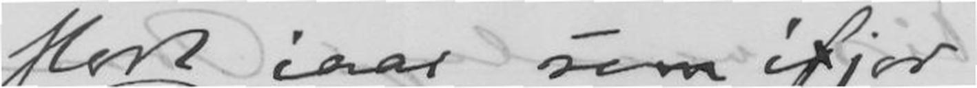stort iaar som ifjor
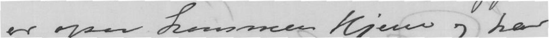er ogsaa kommen Hjem og har
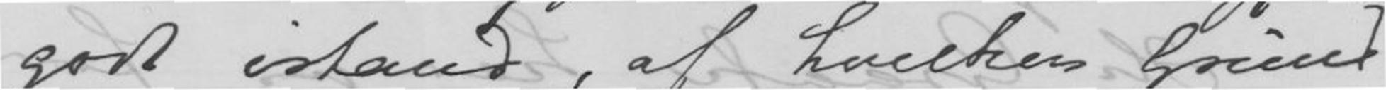godt istand, af hvilken Grund
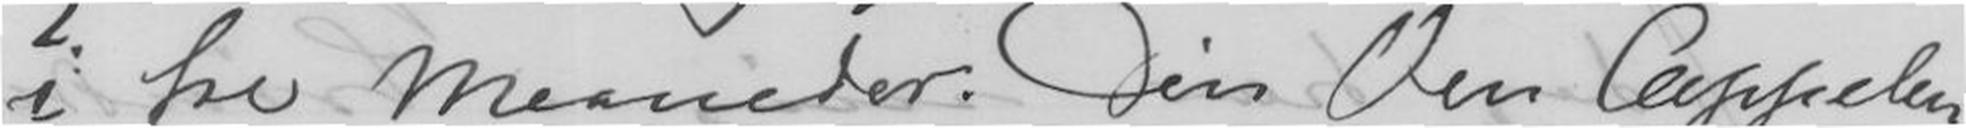i tre Maaneder. Din Ven Cappelen
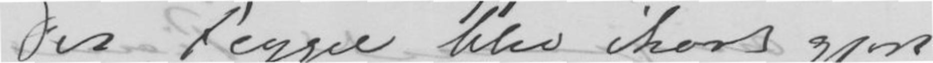Dit Flygel blev ihøst gjort
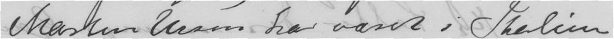Martin Urson har været i Italien
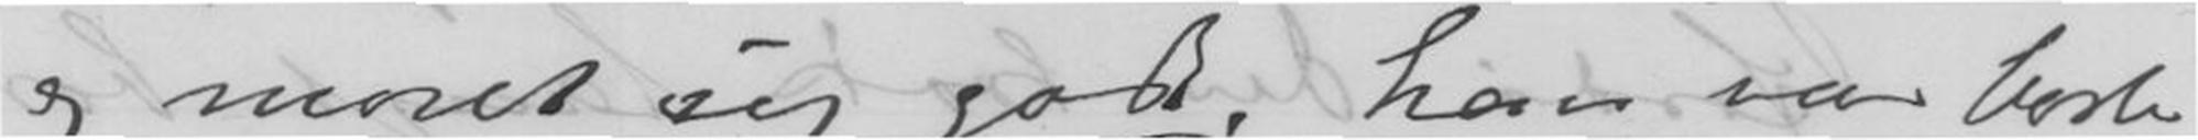og moret sig godt, han var borte
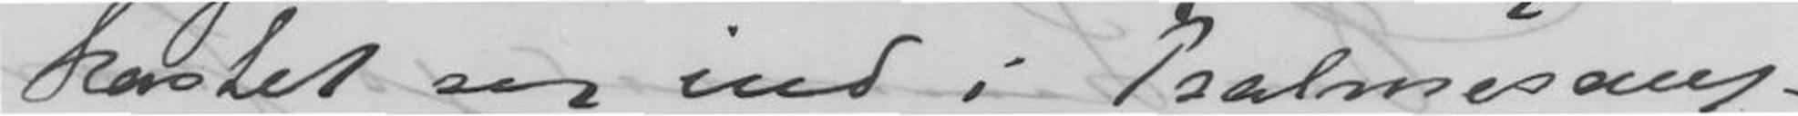kastet sig ind i Psalmesang-
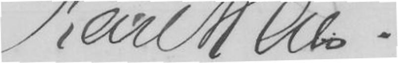Karl Hals.
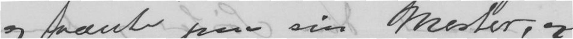og vænte paa sin Mester, og
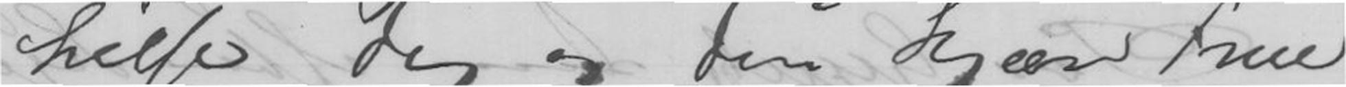hilse Dig og Din kjære Frue
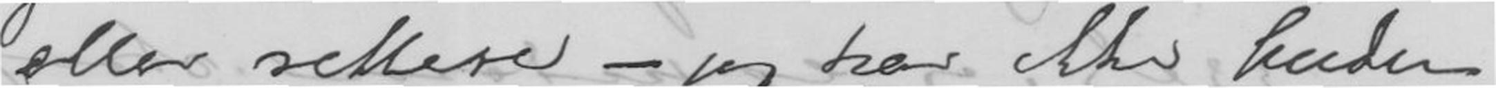eller rettere - jeg har ikke buden
